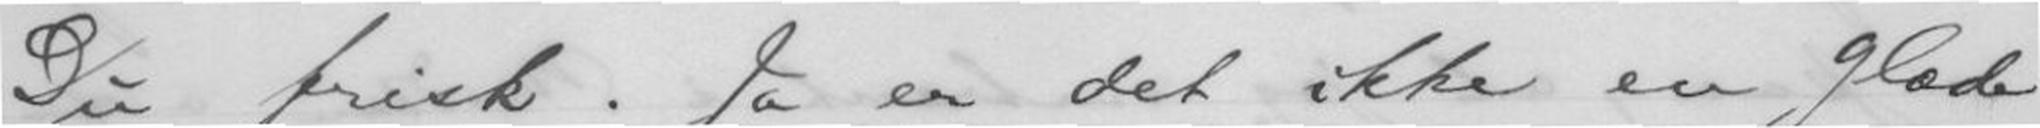Du frisk. Ja er det ikke en Glæde
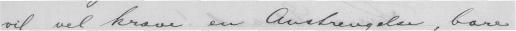vil vel kræve en Anstrengelse, bare
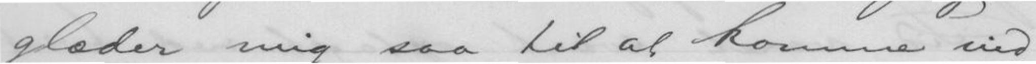glæder mig saa til at komme ind
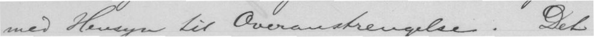med Hensyn til Overanstrengelse. Det
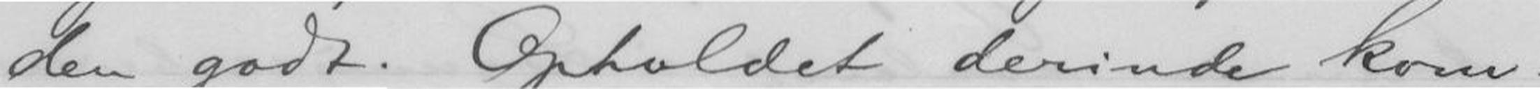den godt. Opholdet derinde kom-
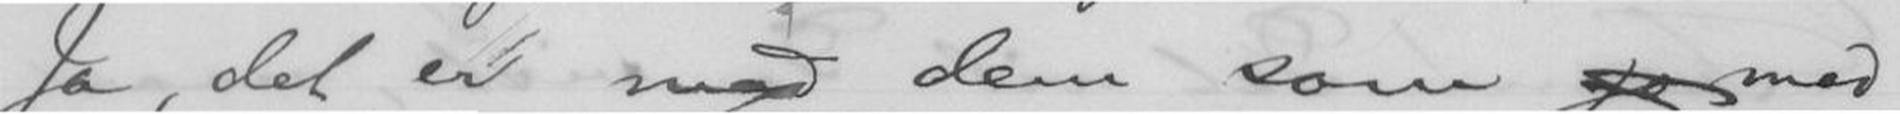Ja, det er med den som jo med
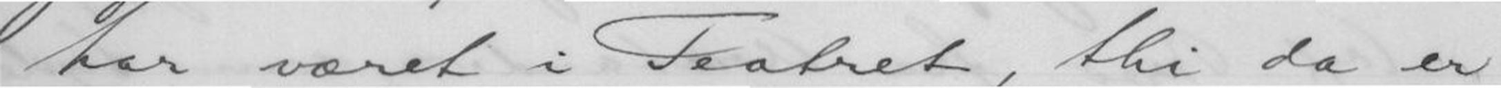har været i Teatret, thi da er
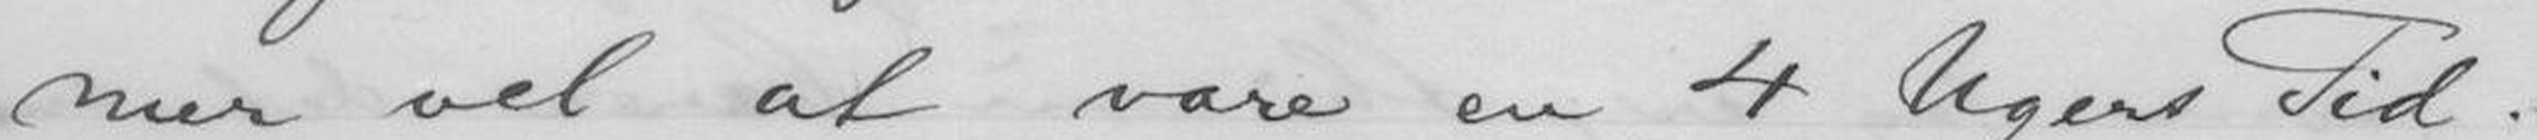mer vel at vare en 4 Ugers Tid.
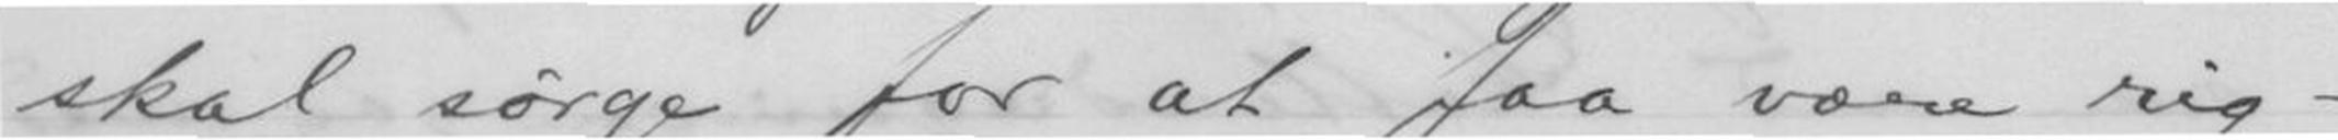skal sørge for at faa være rig-
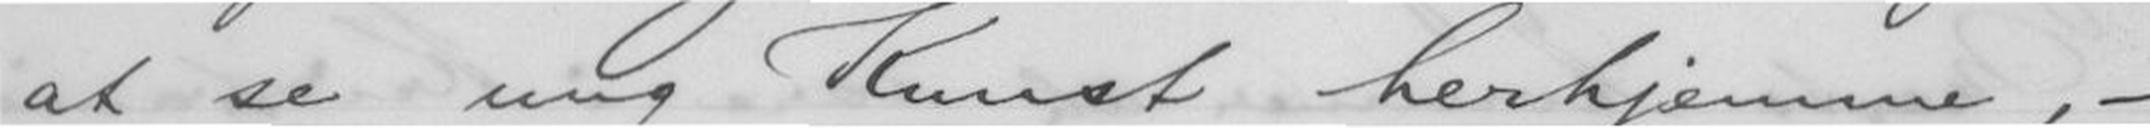at se ung Kunst herhjemme, -
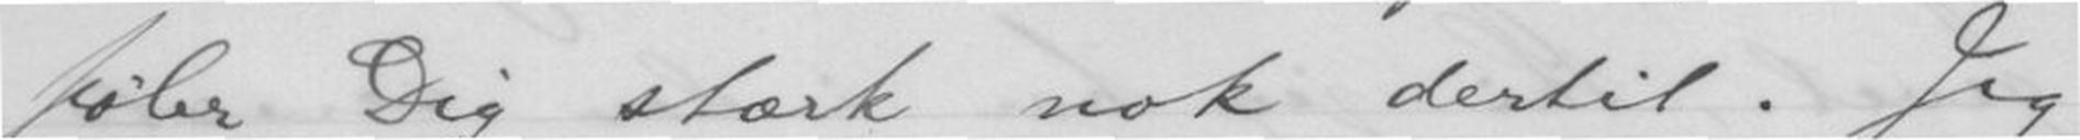føler Dig stærk nok dertil. Jeg
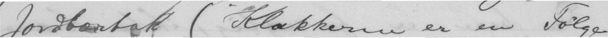Jordbærbak (Klakkerne er en Følge
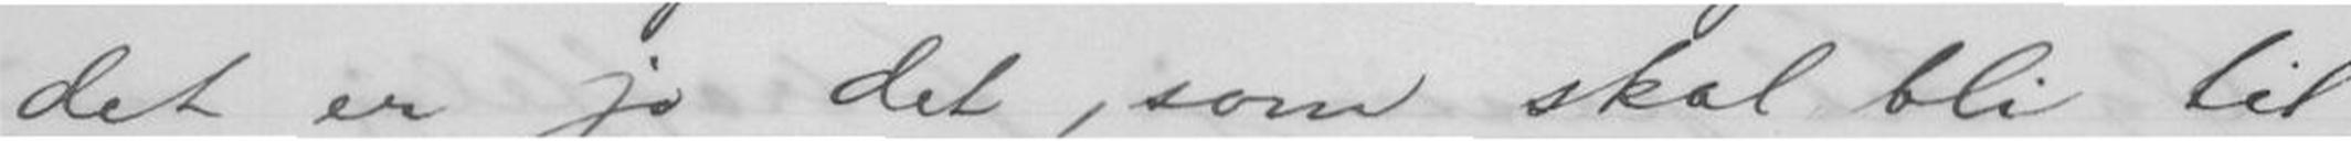det er jo det, som skal bli til
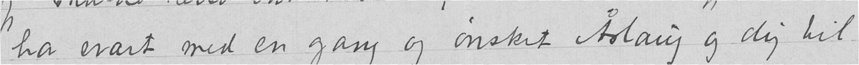ha svart med en gang og ønsket Åslaug og dig til
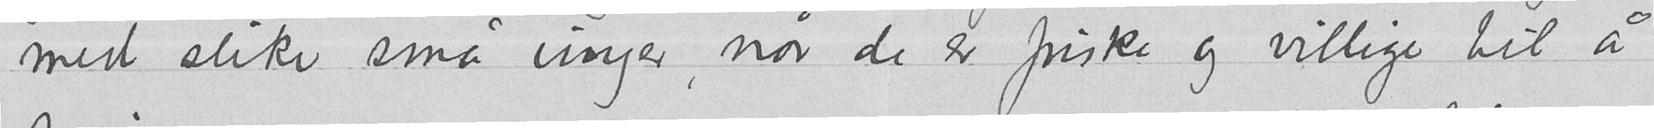med slike små unger, når de er friske og villige til å
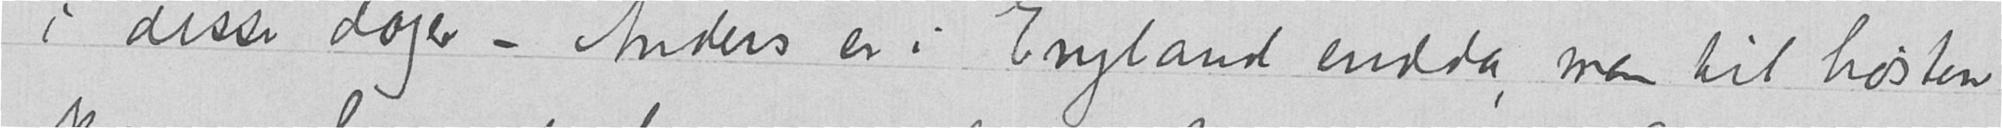i disse dager – Anders er i England endda, men til høsten
